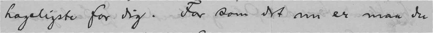hageligste for dig. For som det nu er maa du
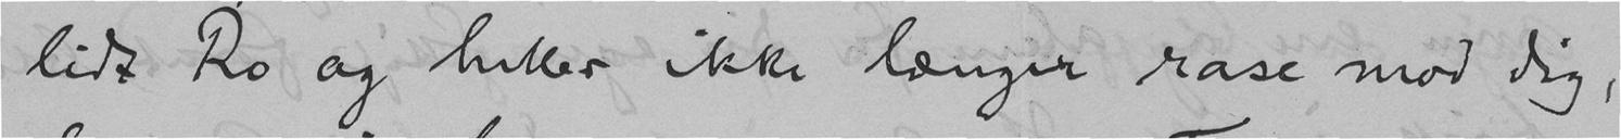lidt Ro og heller ikke længer rase mod dig,
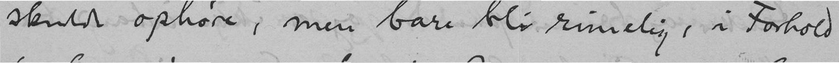skulde ophøre, men bare bli rimelig, i Forhold
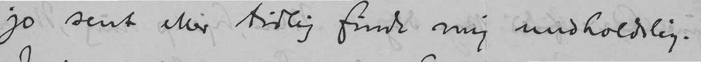jo sent eller tidlig finde mig uudholdelig.
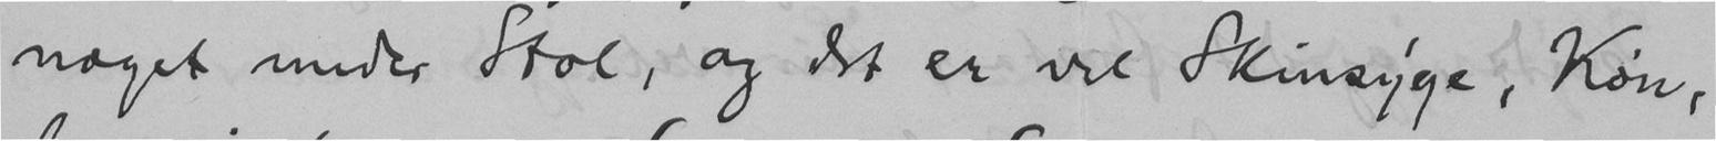noget under Stol, og det er vel Skinsyge, Køn,
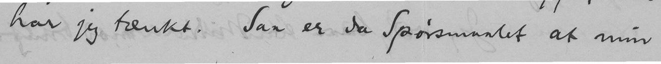har jeg tænkt. Saa er da Spørsmaalet at min
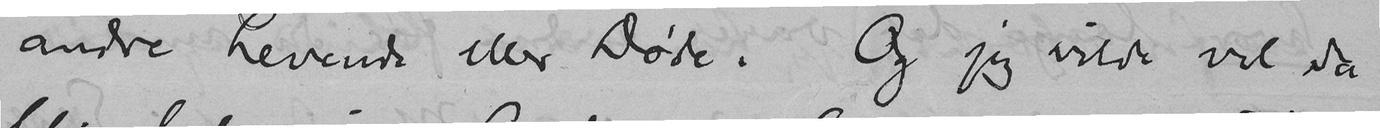andre Levende eller Døde. Og jeg vilde vel da
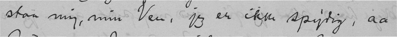staa mig, min Ven, jeg er ikke spydig, aa
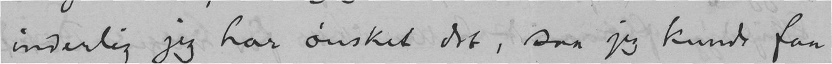inderlig jeg har ønsket det, saa jeg kunde faa
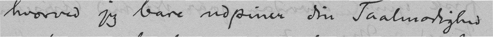hvorved jeg bare udpiner din Taalmodighed
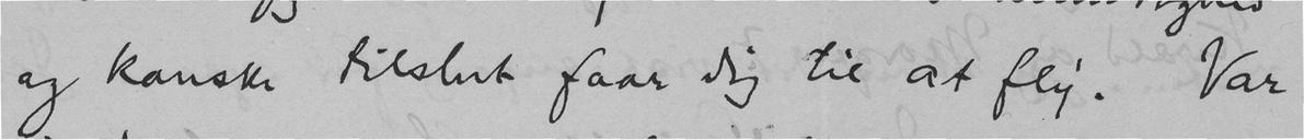og kanske tilslut faar dig til at fly. Var
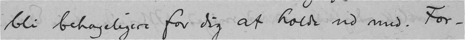bli behageligere for dig at holde ud med. For-
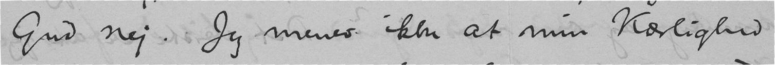Gud nej. Jeg mener ikke at min Kærlighed
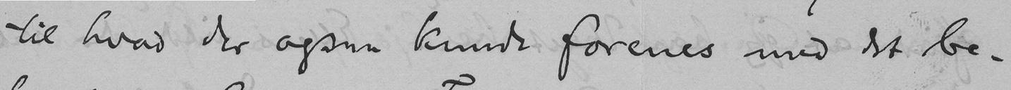til hvad der ogsaa kunde forenes med det be-
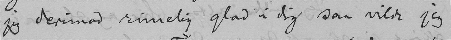jeg derimod rimelig glad i dig saa vilde jeg
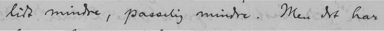lidt mindre, passelig mindre. Men det har
